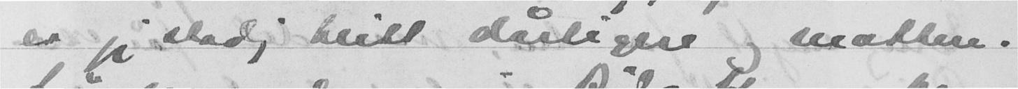er jeg stadig blitt dårligere og mattere.
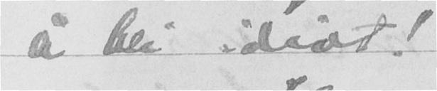å bli idiot!
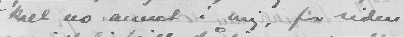kalt no annet i mig, for siden
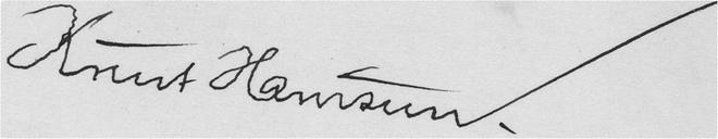Knut Hamsun.
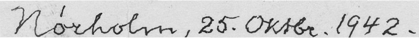Nørholm, 25. Oktbr. 1942.
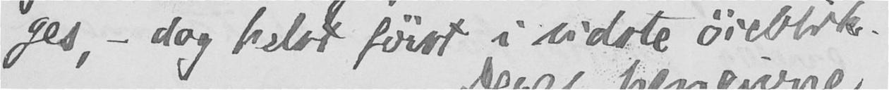ges, - dog helst først i sidste øieblik.
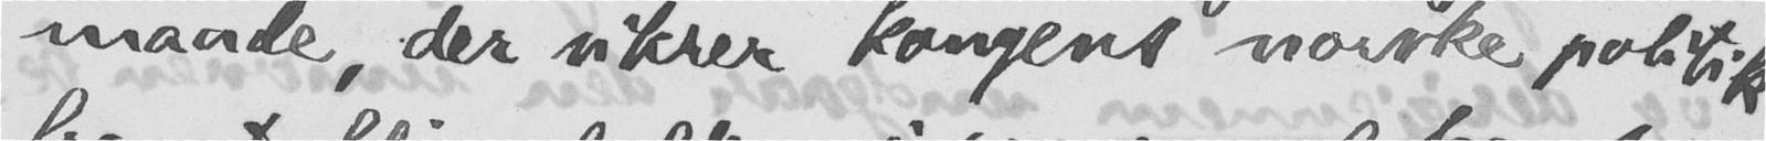maade, der sikrer kongens norske politik
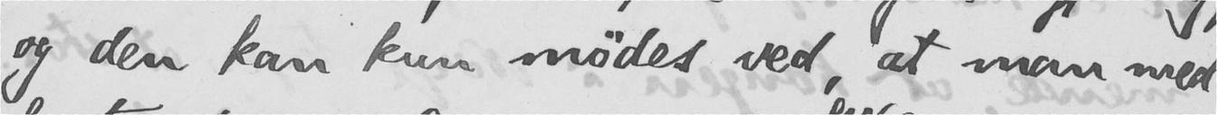og den kan kun mødes ved, at man med
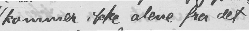kommer ikke alene fra det
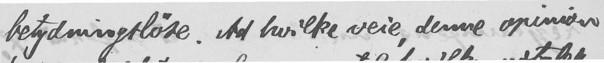betydningsløse. Ad hvilke veie, denne opinion
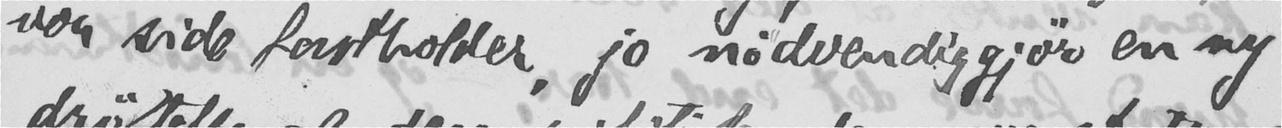vor side fastholder, jo nødvendiggjør en ny
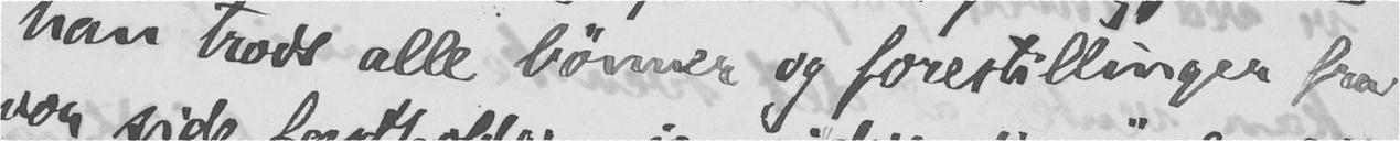han trods alle bønner og forestillinger fra
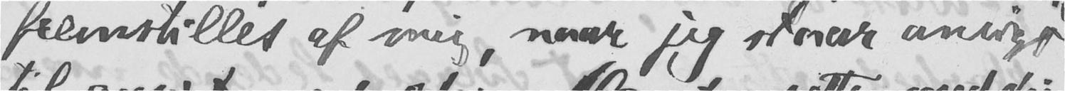fremstilles af mig, naar jeg staar ansigt
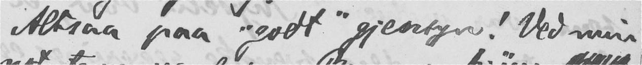Altsaa paa "godt" gjensyn! Ved min
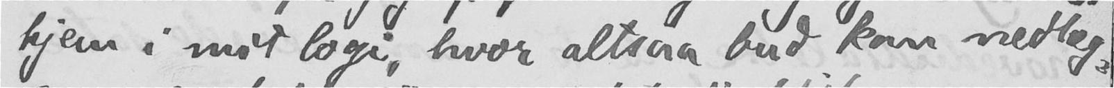hjem i mit logi, hvor altsaa bud kan nedlæg-
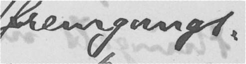fremgangs-
